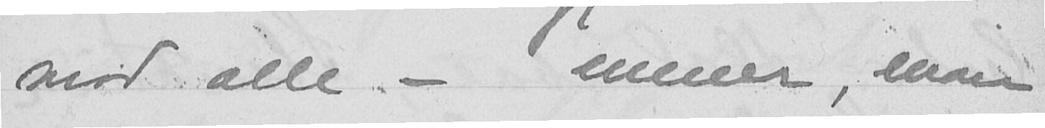mod alle - mener, man
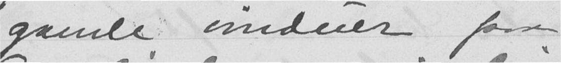gamle vinduer paa
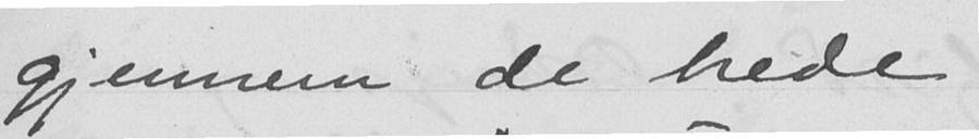gjennem de brede
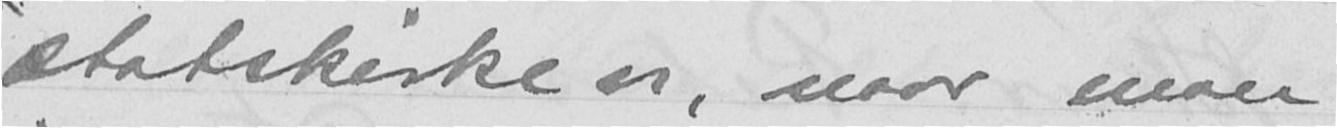statskirken, naar man
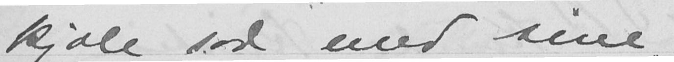kjole sad med sine
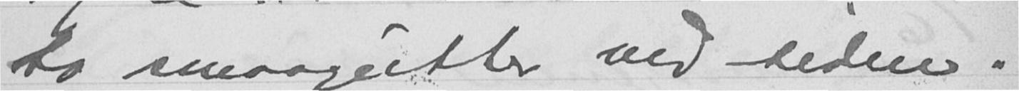to smaagutter ved siden.
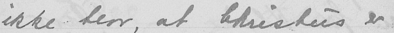ikke tror, at Christus er
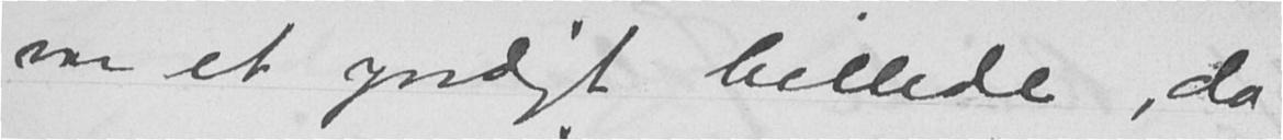var et yndigt billede, da
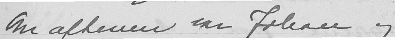Om aftenen var Johan og
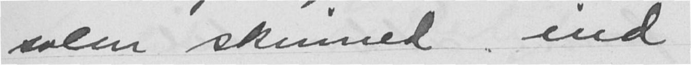solen skinnet ind
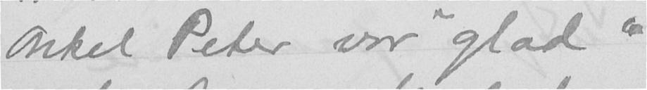Onkel Peter var "glad"
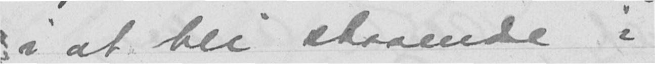i at bli staaende i
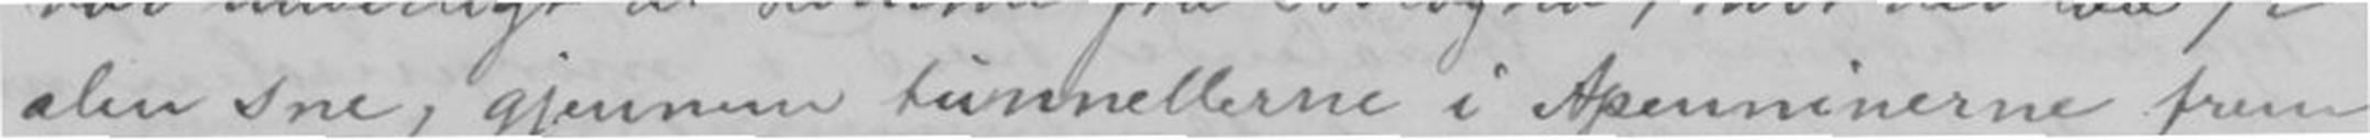alen sne, gjennum tunnellerne i Apenninerne frem
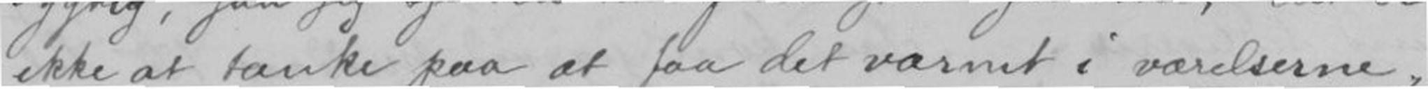ikke at tænke paa at faa det varmt i værelserne,
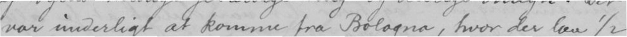var underligt at komme fra Bologna hvor der laa 1/2
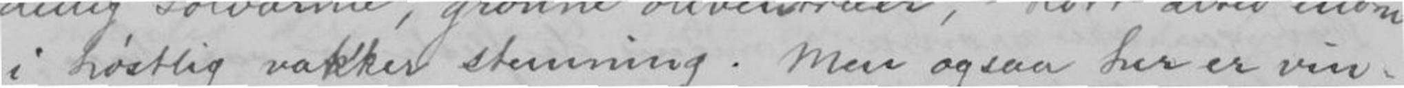i høstlig vakker stemning. Men ogsaa her er vin-
